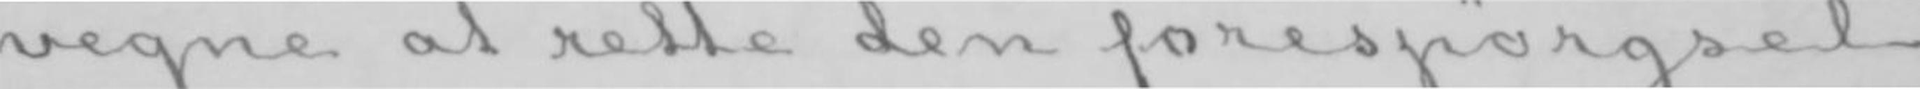vegne at rette den forespørgsel
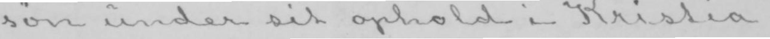søn under sit ophold i Kristia-
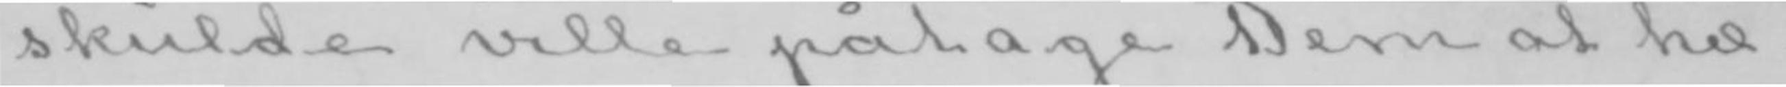skulde ville påtage Dem at hæ-
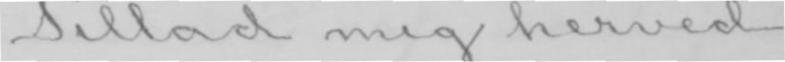Tillad mig herved
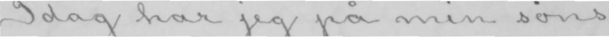Idag har jeg på min søns
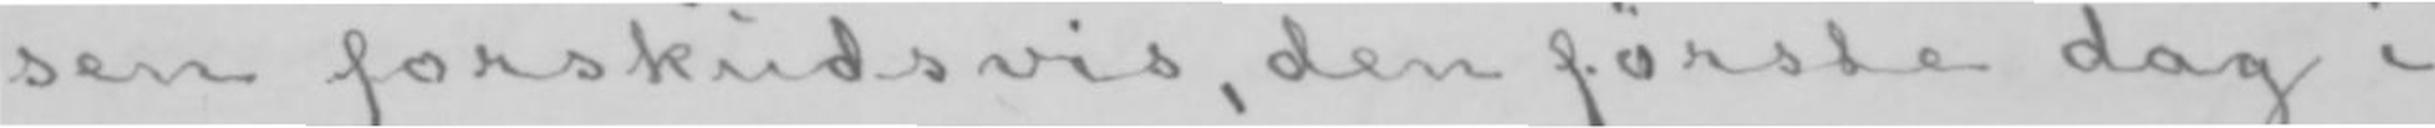sen forskudsvis, den første dag i
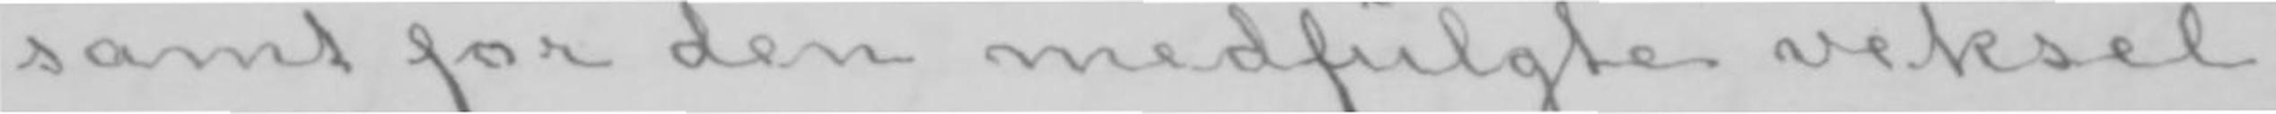samt for den medfulgte veksel.
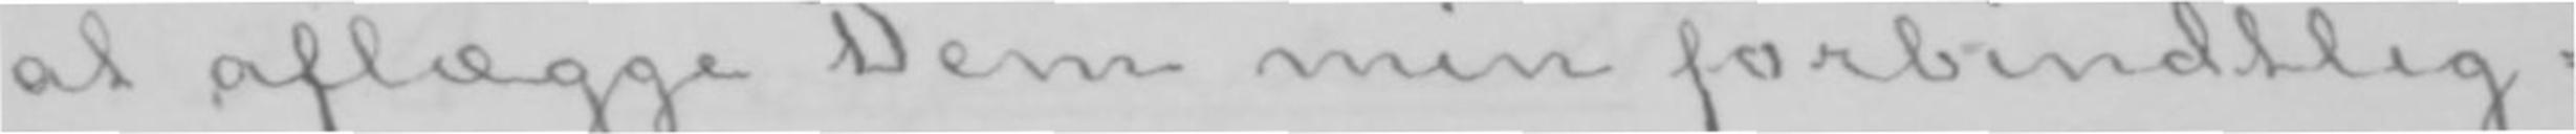at aflægge Dem min forbindtlig-
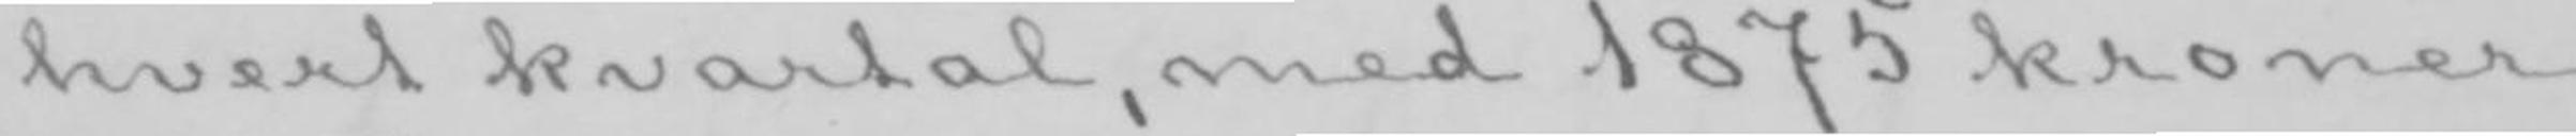hvert kvartal, med 1875 kroner.
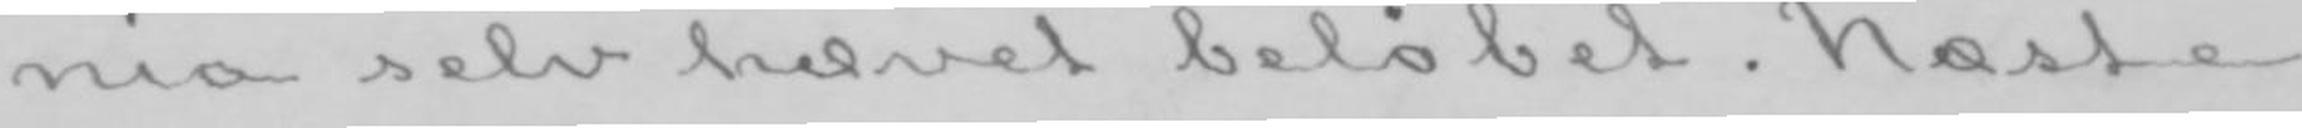nia selv hævet beløbet. Næste
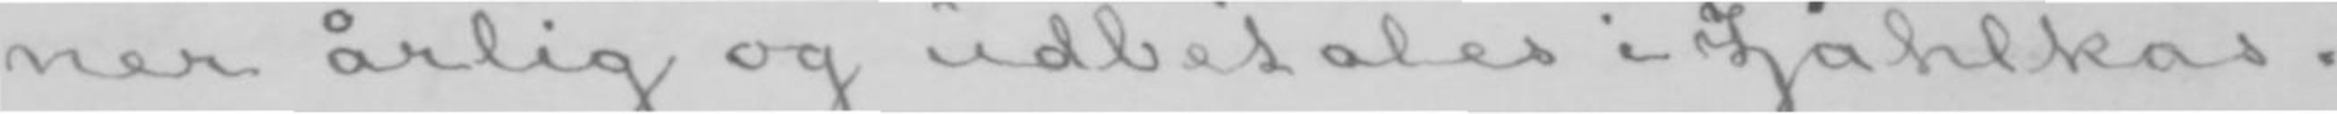ner årlig og udbetales i Zahlkas-
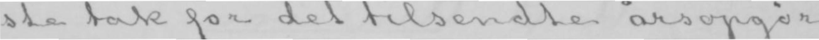ste tak for det tilsendte årsopgør
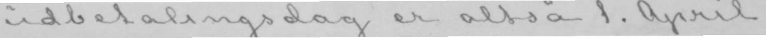udbetalingsdag er altså 1. April.
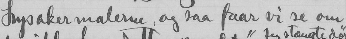Lysakermalerne, og saa faar vi se om
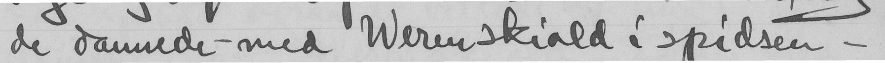de dannede - med Werenskiold i spidsen -
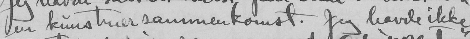en kunstmersammenkomst. Jeg havde ikke
